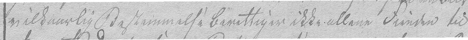vilkaarlig Bestemmelse berettiger ikke allene Fienden til
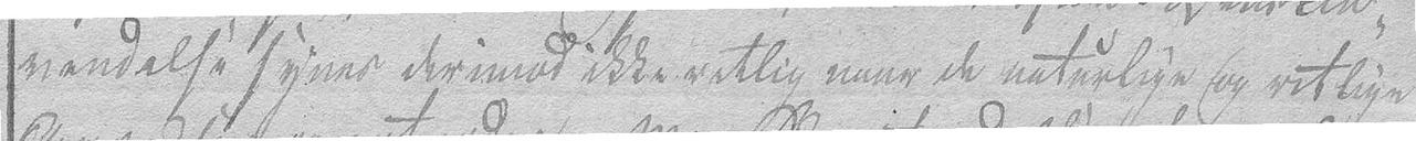vendelse synes derimod ikke retlig naar de naturlige og retlige
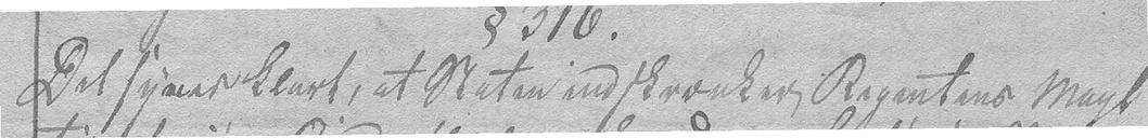Det synes klart, at Staten indskrænker Regentens Magt
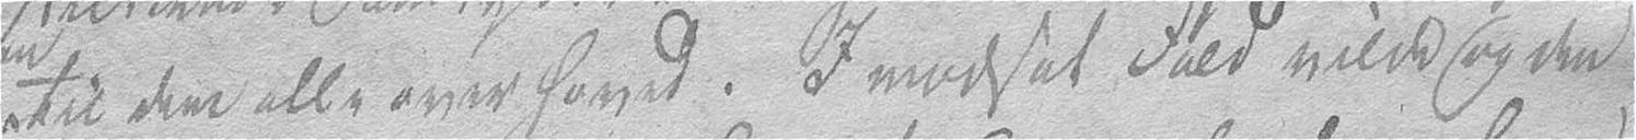til dem alle overhoved. I modsat Fald vilde og den
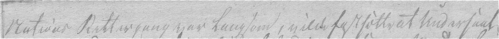Nations Rettergang var langsom, vilde fastsatte at Undersaat-
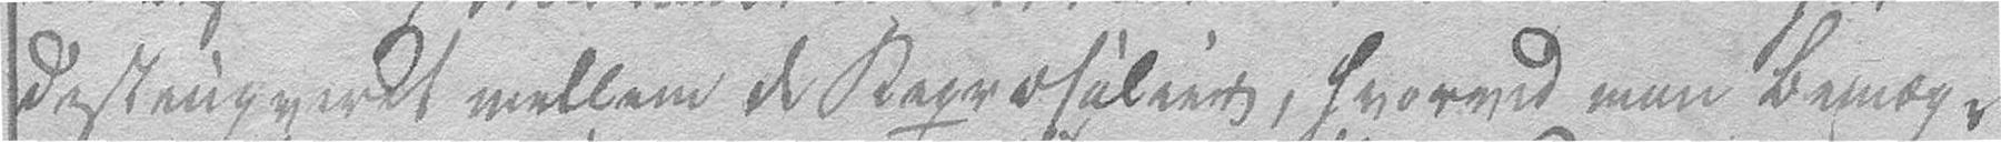distingveret mellem de Repræsalier, hvorved man bemæg-
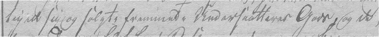tigede sig og solgte fremmede Undersaatteres Gods, og de
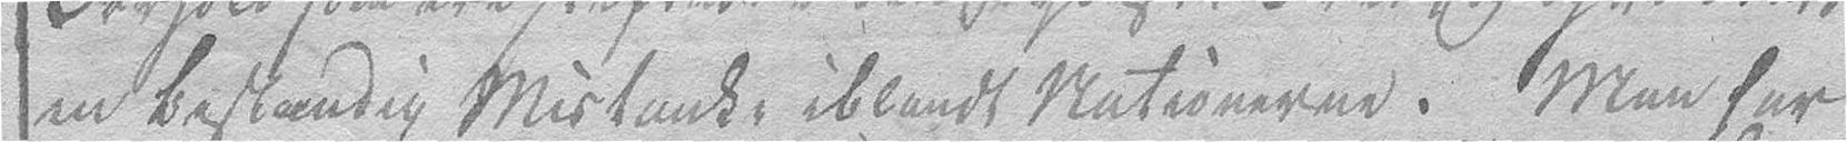en bestandig Mistanke iblandt Nationerne. Man har
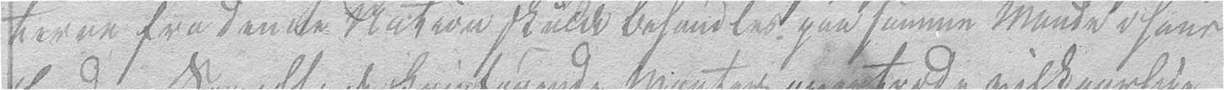terne fra denne Nation skulde belandles paa samme Maade i hans
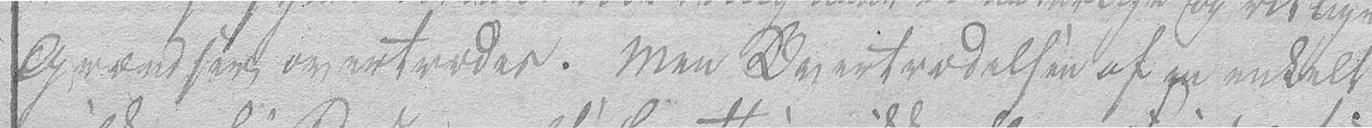Grændser overtrædes. Men Overtrædelsen af en enkelt
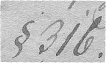§ 316.
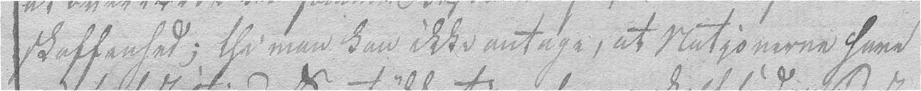skaffenhed; thi man kan ikke antage, at Nationerne have
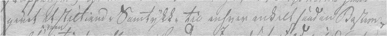givet et stiltiende Samtykke til enhver enkelt saadan Bestem-
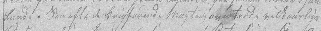Land. Saa ofte de krigførende Magter overtræde vilkaarlige
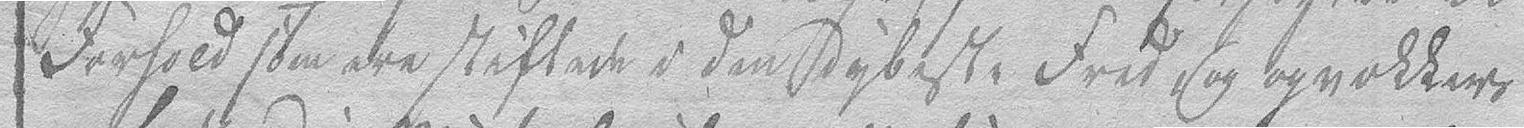Forhold som ere stiftede i den dybeste Fred, og opvækker
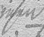men
